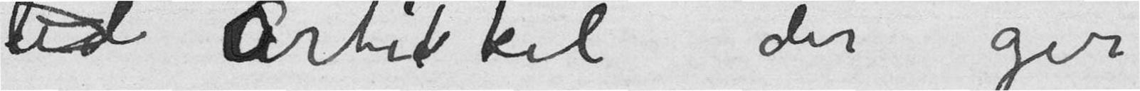lid artikkel der gir
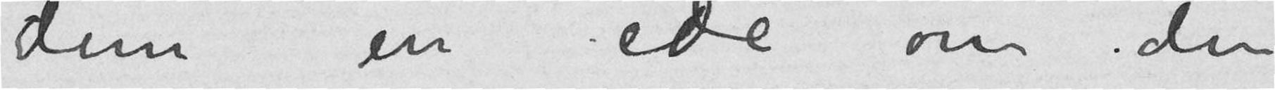dem en ide om den
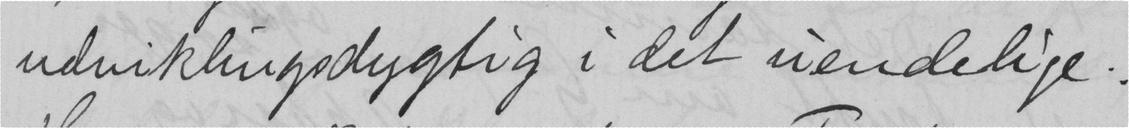udviklingsdygtig i det uendelige.
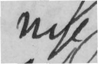nye
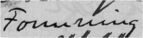Formning
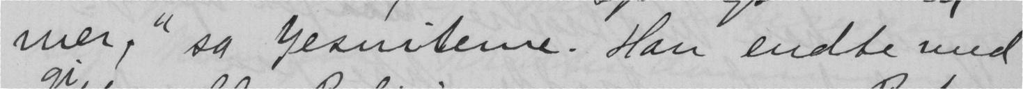mer," sa jesuiterne. Han endte med
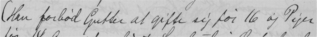Han forbød Gutter at gifte sig før 16 og Piger
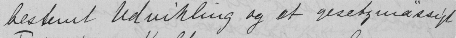bestemt Udvikling og et gesetzmässigt
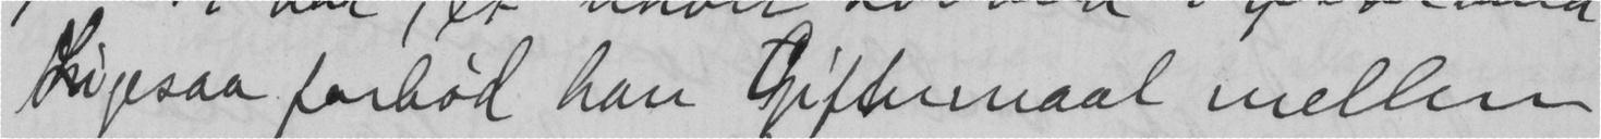Ligesaa forbød han Giftermaal mellem
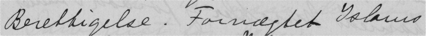Berettigelse. Fornægtet Islams
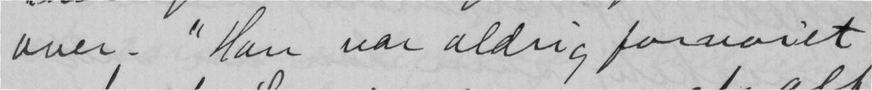over. "Han var aldrig fornøiet
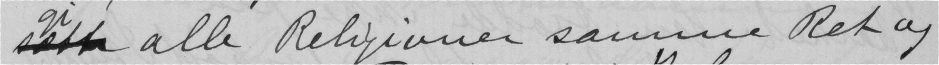alle Religioner samme Ret og
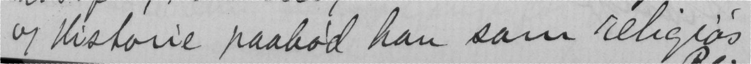og Historie paabød han som religiøs
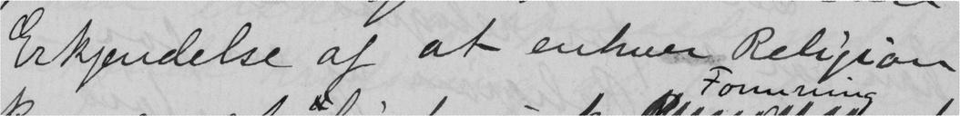Erkjendelse af at enhver Religion
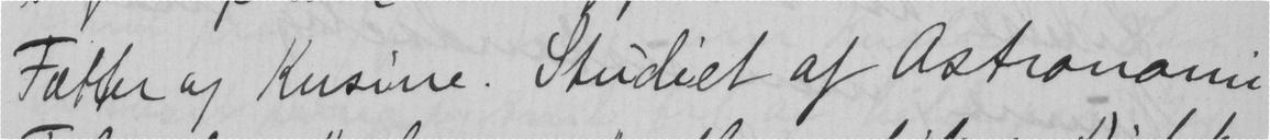Fætter og Kusine. Studiet af Astronomi
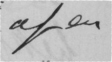af en
